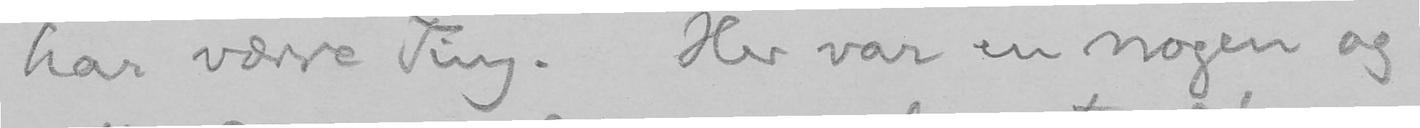har værre Ting. Her var en nogen og
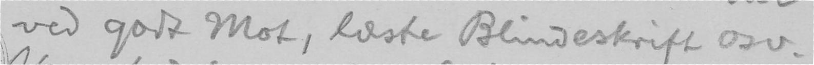ved godt Mot, læste Blindeskrift osv.
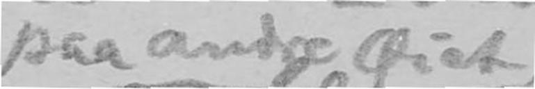paa andre Øiet
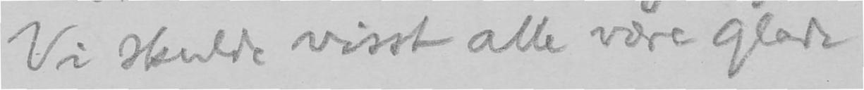Vi skulde visst alle være glade
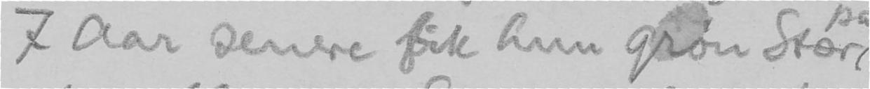7 Aar senere fik hun grøn Stær
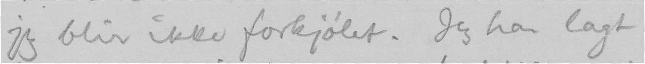jeg blir ikke forkjølet. Jeg har lagt
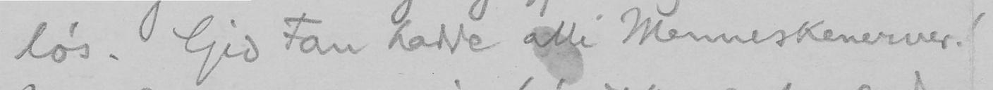løs. Gid Fan hadde alle Menneskenerver!
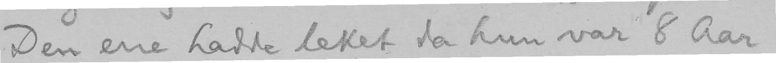Den ene hadde leket da hun var 8 Aar
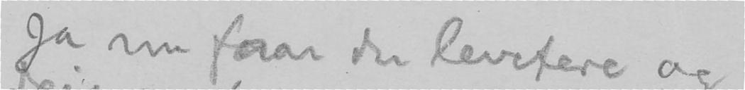Ja nu faar du levetere og
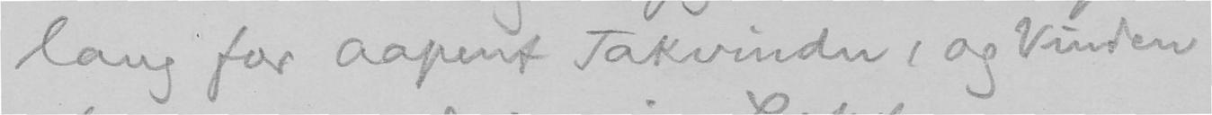lang for aapent Takvindu, og Vinden
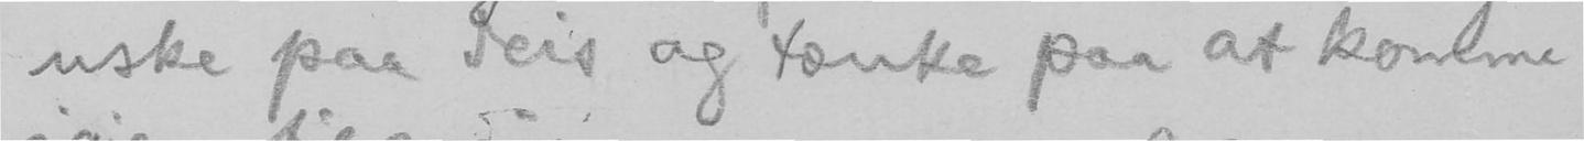uske paa deis og tænke paa at komme
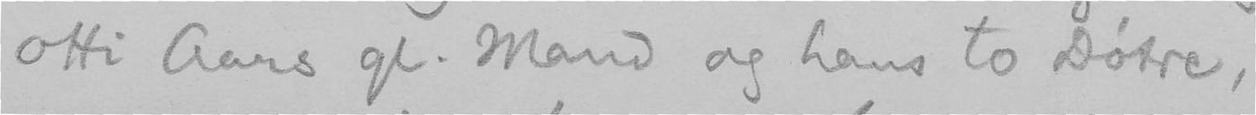otti Aars gl. Mand og hans to Døtre,
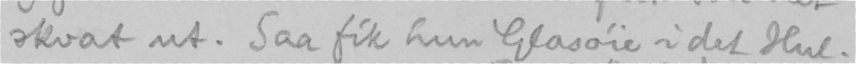skvat ut. Saa fik hun Glasøie i det Hul.
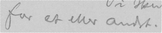for et eller andet.
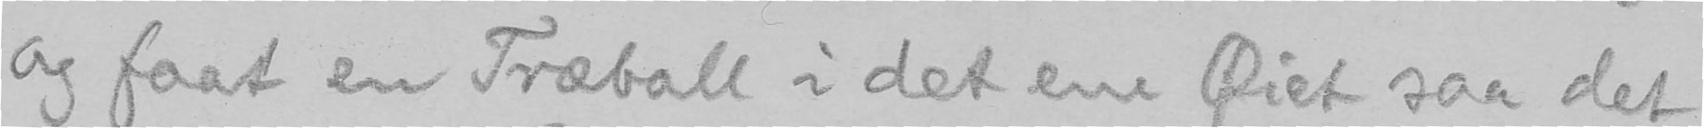og faat en Træball i det ene Øiet saa det
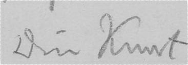Din Knut
